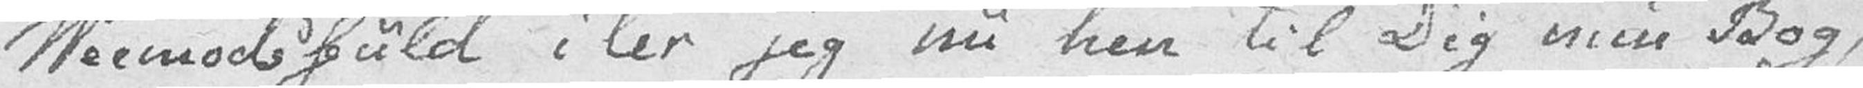Veemodsfuld iler jeg nu hen til Dig min Bog,
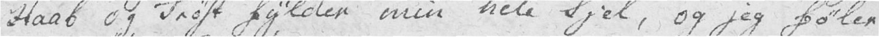Haab og Trøst fylder min hele Sjel, og jeg føler
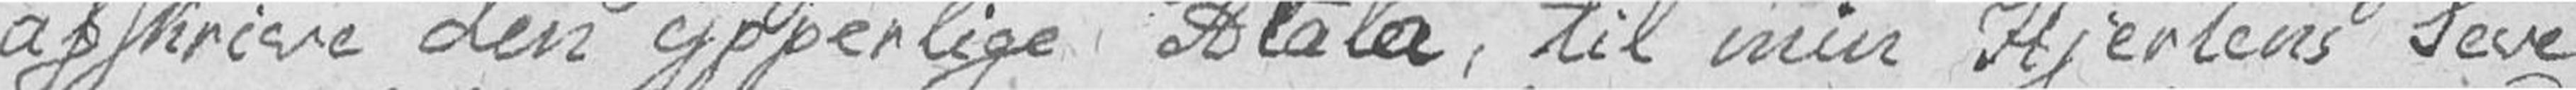afskrive den ypperlige Atala, til min Hjertens Seve
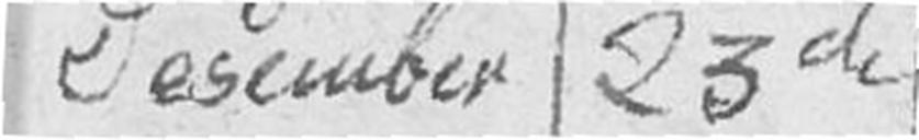Desember 23de
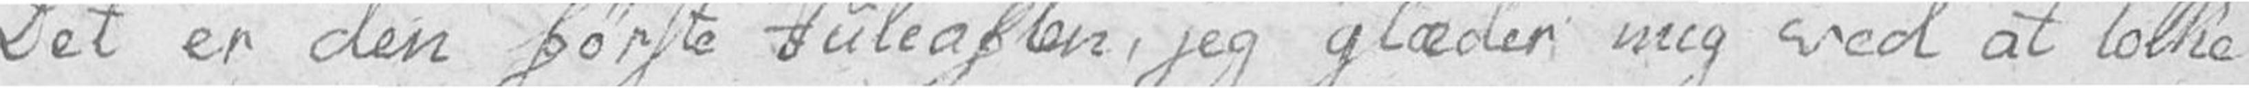Det er den første Juleaften, jeg glæder mig ved at tolke
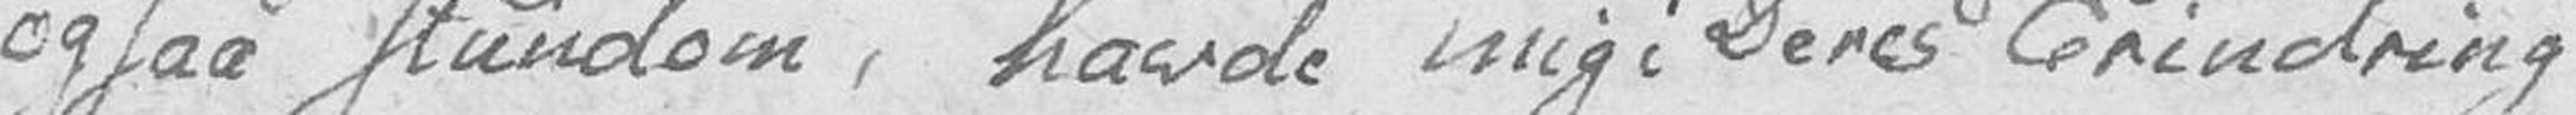ogsaa stundom, havde mig i Deres Erindring
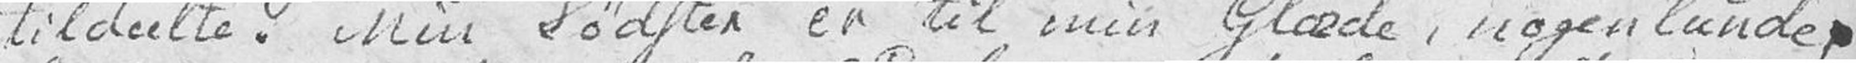tildeelte. Min Sødster er til min Glæde, nogenlunde,
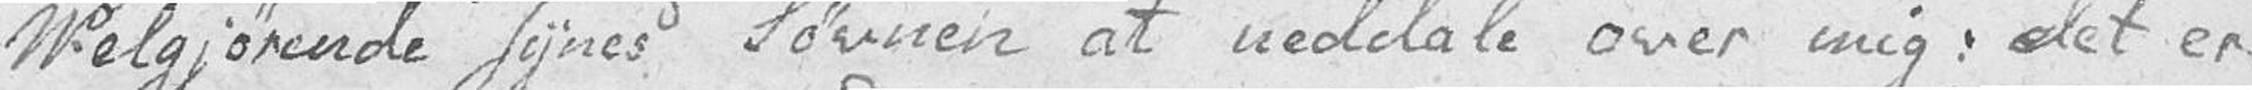Velgjørende synes Søvnen at neddale over mig: det er
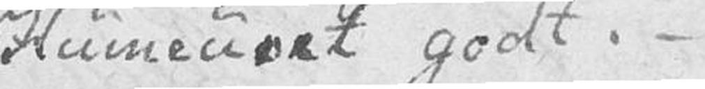Humeuret godt. –
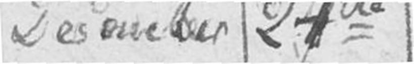Desember 24de
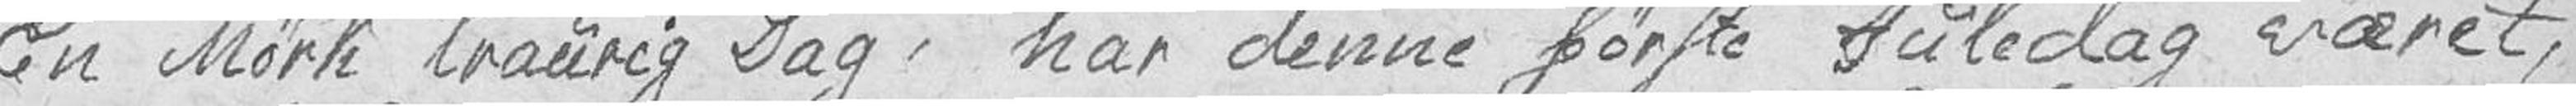En Mørk traurig Dag, har denne første Juledag været,
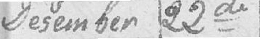Desember 22de
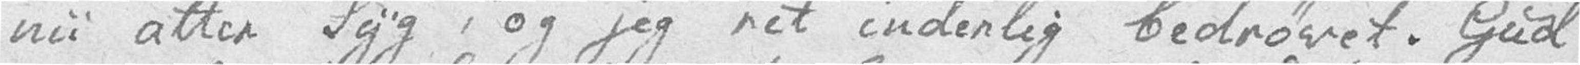nu atter Syg, og jeg ret inderlig bedrøvet. Gud
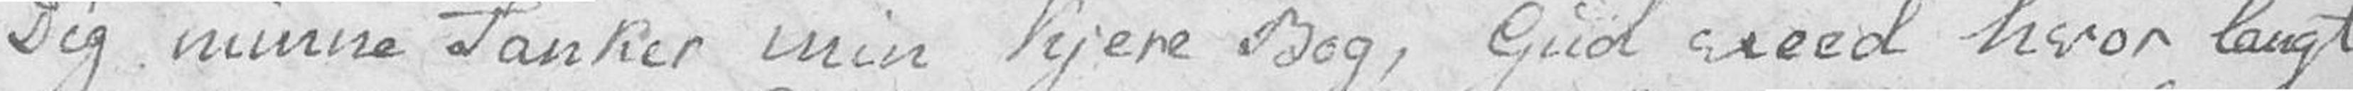Dig minne Tanker min kjere Bog, Gud veed hvor langt
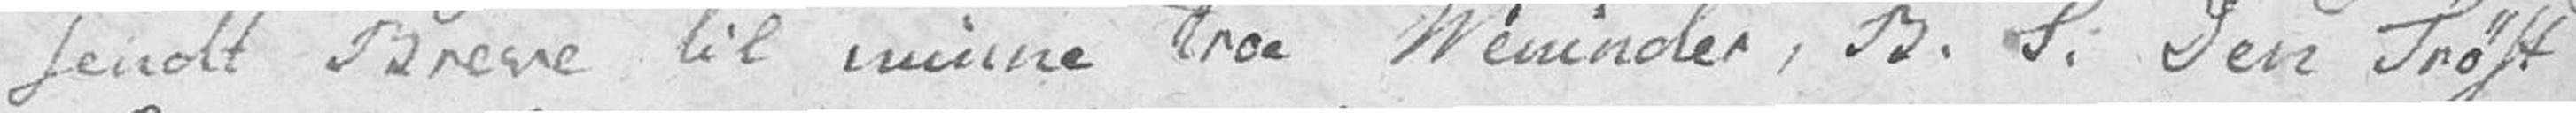sendt Breve til minne troe Veninder, B. S. Den Trøst
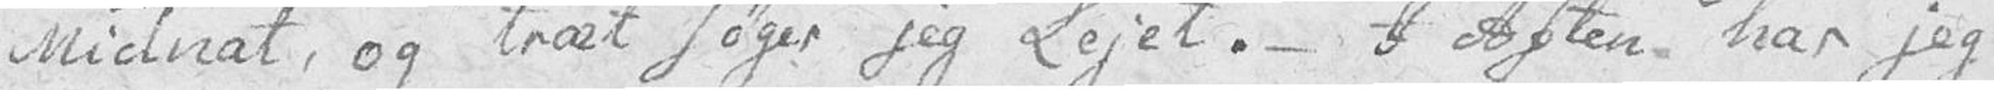Midnat, og træt søger jeg Lejet. – I Aften har jeg
